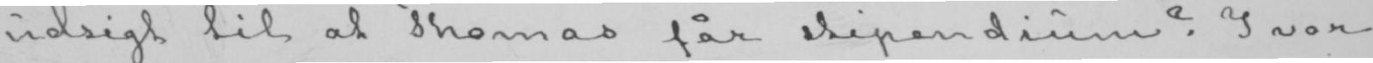udsigt til at Thomas får stipendium? I vor
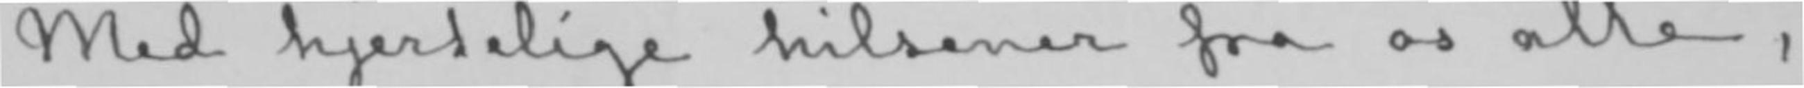Med hjertelige hilsener fra os alle,
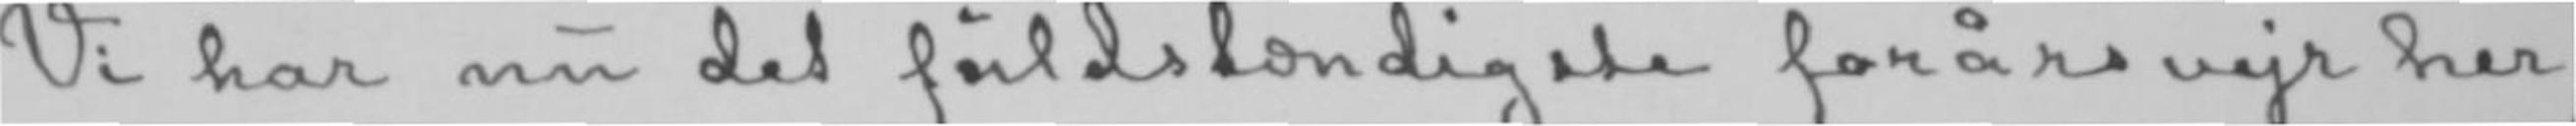Vi har nu det fuldstændigste forårsvejr her,
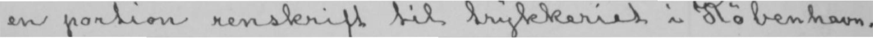en portion renskrift til trykkeriet i København.
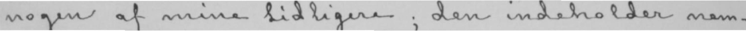nogen af mine tidligere; den indeholder nem-
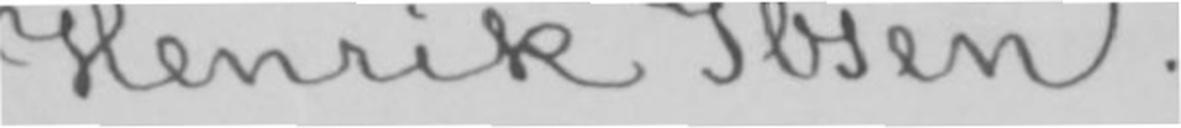Henrik Ibsen.
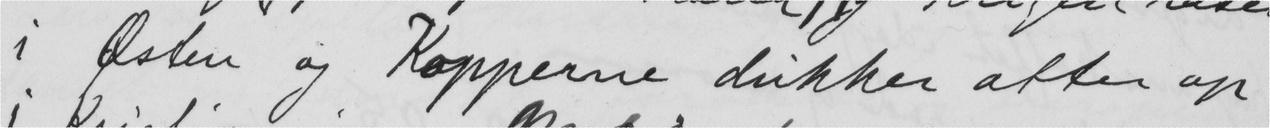i Østen og Kopperne dukker atter op
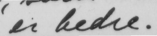er bedre.
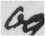og
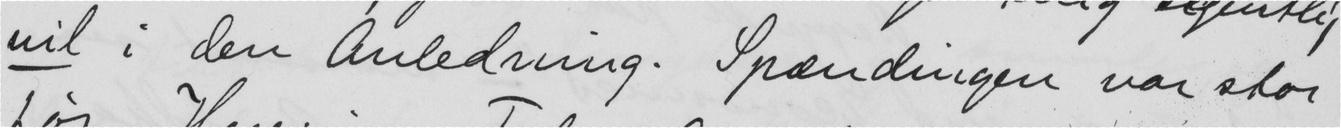vil i den Anledning. Spændingen var stor
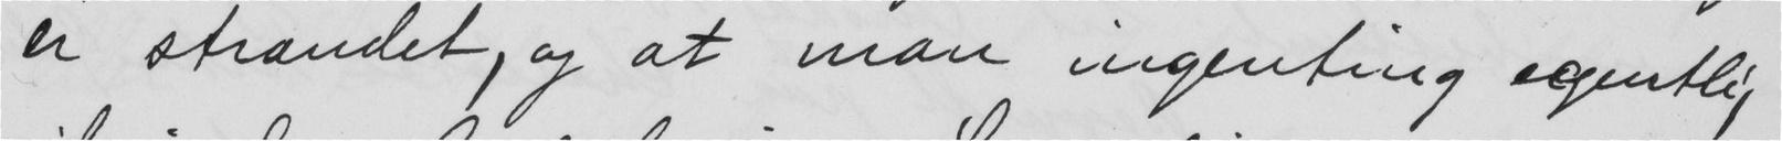er strandet, og at man ingenting egentlig
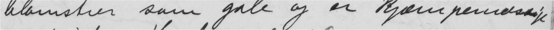blomstrer som gale og er kjæmpemæssige
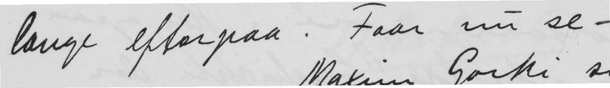lange efterpaa. Faar nu se -
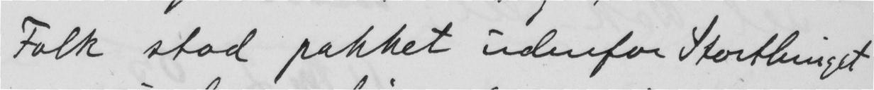Folk stod pakket udenfor Storthinget
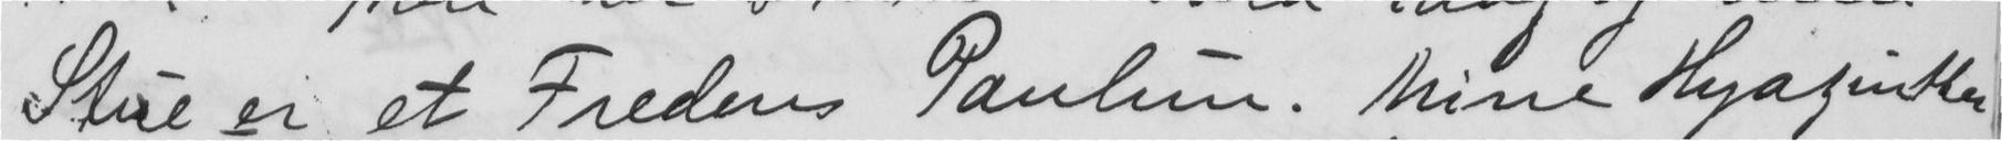Stue er et Fredens Panlun. Mine Hyazinther
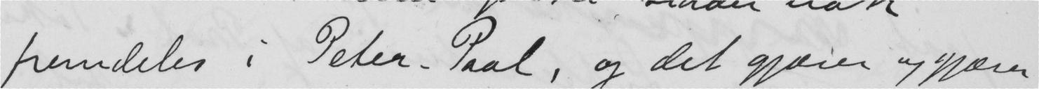fremdeles i Peter-Paal, og det gjærer og gjærer,
